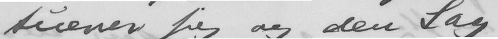tuerer sig og den Sag
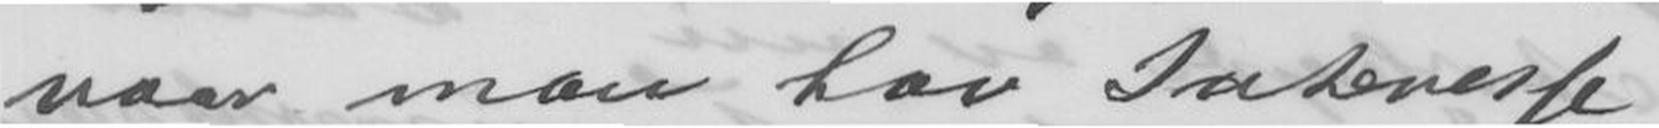naar man har Indtresse
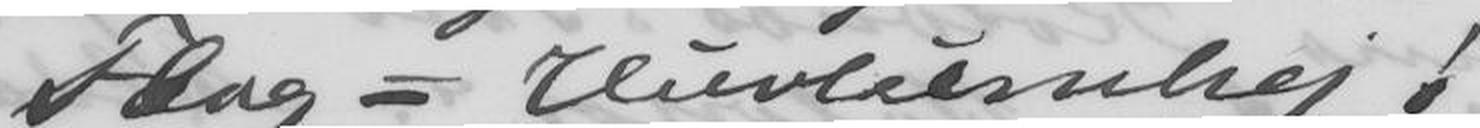Flag = Hurlumhej!
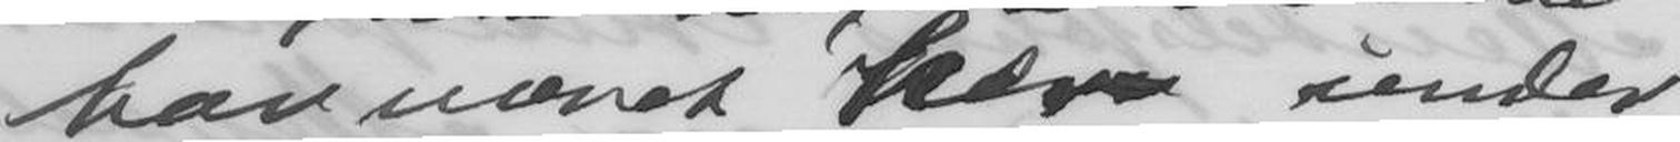har været her under
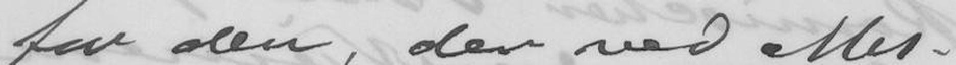for den, der ved Mis-
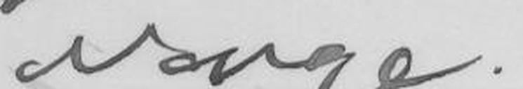Norge!
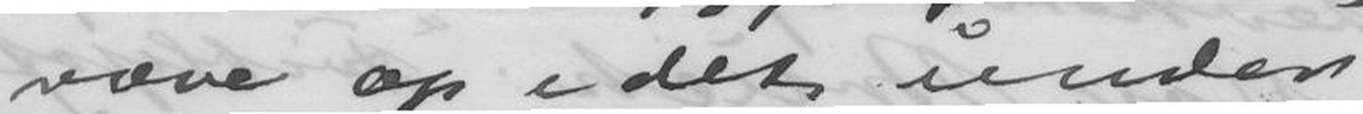være op i det under
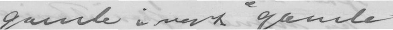gamle i vort gamle
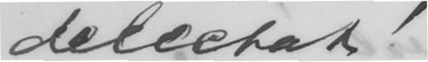delectat! -
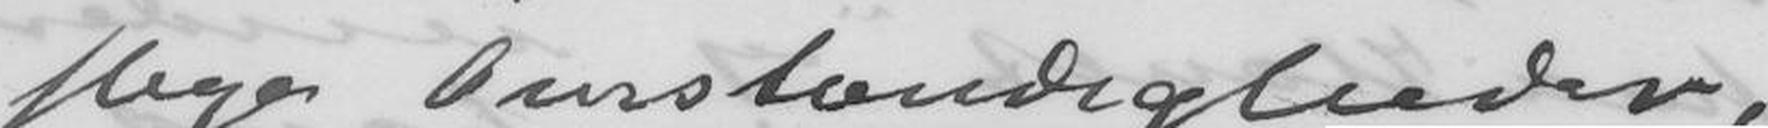slige Omstændigheder,
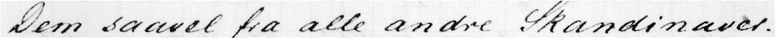Dem saavel fra alle andre Skandinaver.
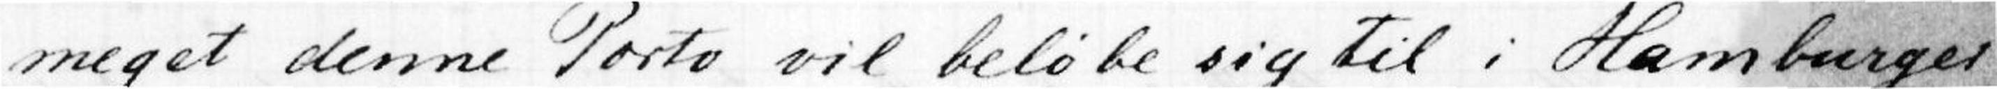meget denne Porto vil beløbe sig til i Hamburges
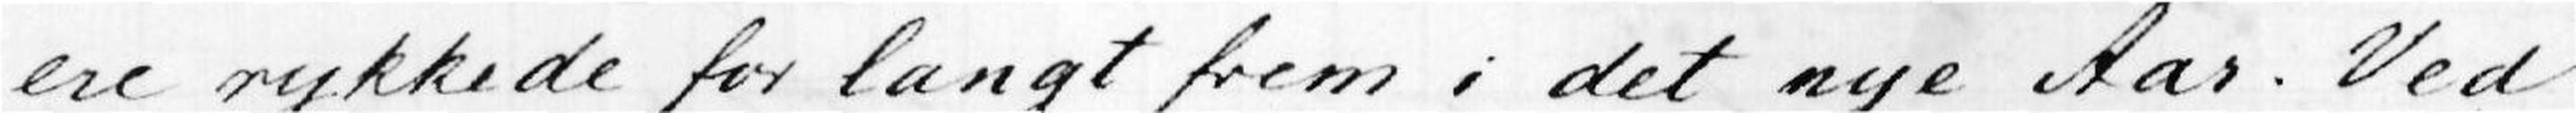ere rykkede for langt frem i det nye Aar. Ved
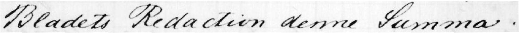Bladets Redaktion denne Summa.
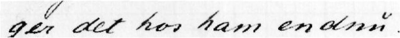ger det hos ham endnu.
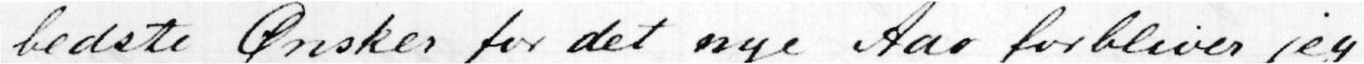bedste Ønsker for det nye Aar forbliver jeg
